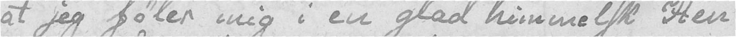at jeg føler mig i en glad himmelsk Hen
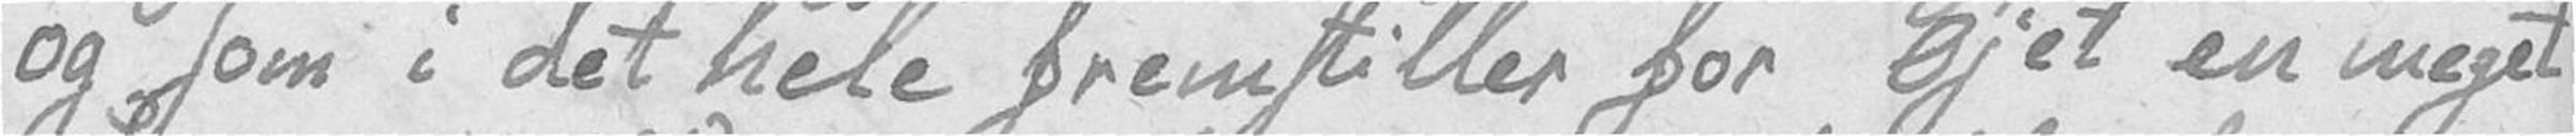og som i det hele fremstiller for Øjet en meget
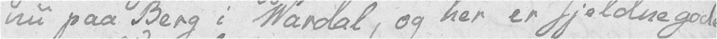nu paa Berg i Vardal, og her er sjeldne gode
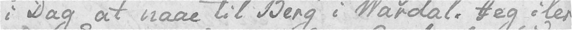i Dag at naae til Berg i Vardal. Jeg iler
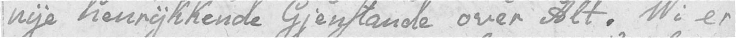nye henrykkende Gjenstande over Alt. Vi er
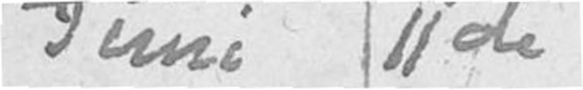Juni 11de
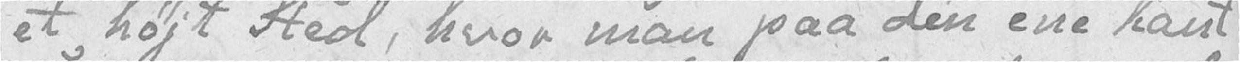et højt Sted, hvor man paa den ene Kant
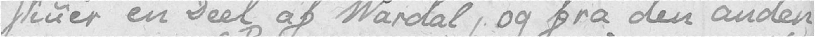skuer en Deel af Vardal, og fra den anden
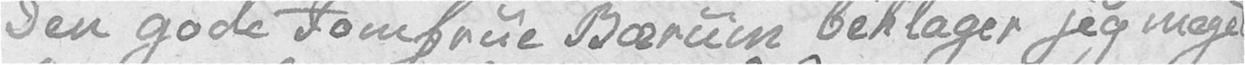Den gode Jomfrue Bærum beklager jeg meget
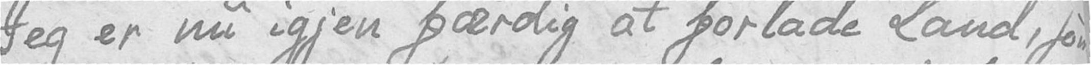Jeg er nu igjen færdig at forlade Land, som
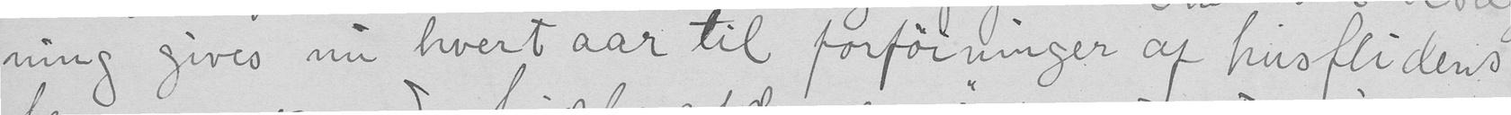ning gives nu hvert aar til forførninger af husflidens
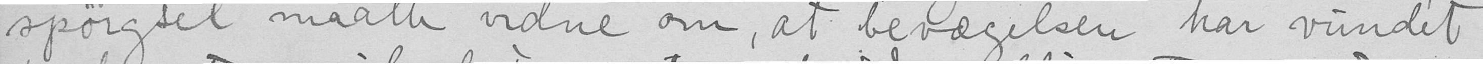spørgsel maatte vidne om, at bevægelsen har vundet
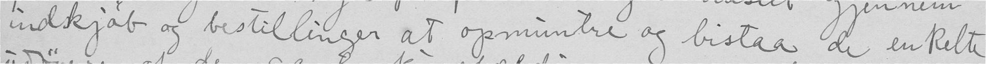indkjøb og bestillinger at opmunre og bistaa de enkelte
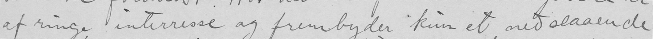af ringe interresse og frembyder kun et nedslaaende
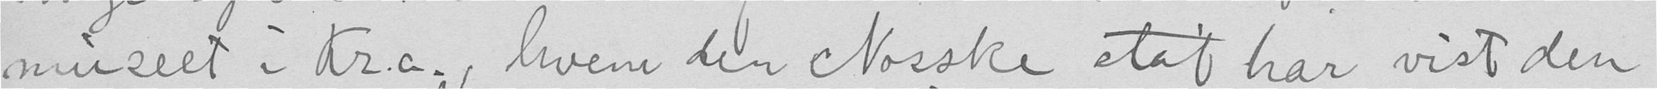museet i Kr.a., hvem den Norske etat har vist den
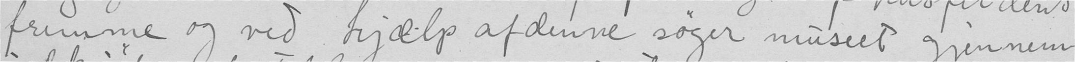fremme og ved hjælp af denne søger museet gjennem
